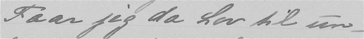Faar jeg da Lov til un-
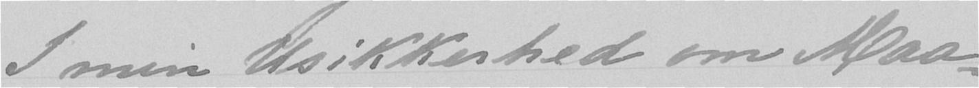I min Usikkerhed om Maa-
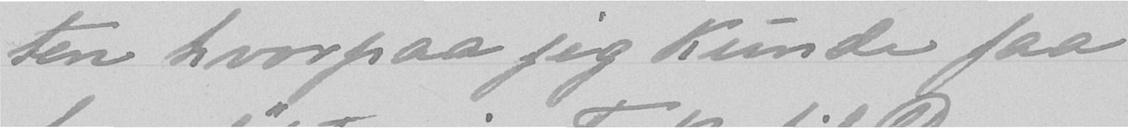ten hvorpaa jeg kunde faa
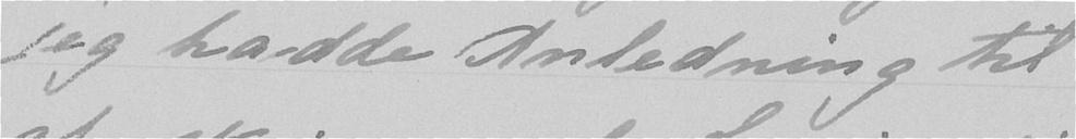jeg hadde Anledning til
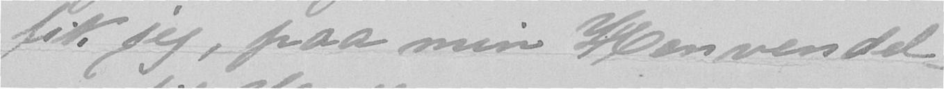fik jeg, paa min Henvendel-
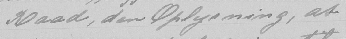Raad, den Oplysning, at
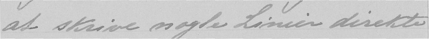at skrive nogle Linier direkte
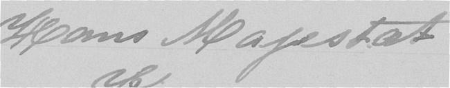Hans Majestæt
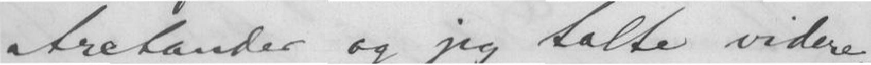Arctander og jeg talte videre,
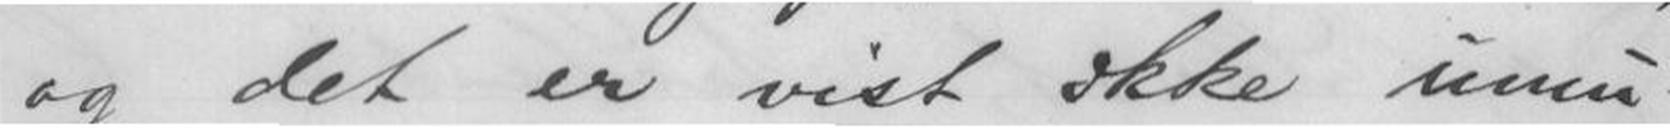og det er vist ikke umu-
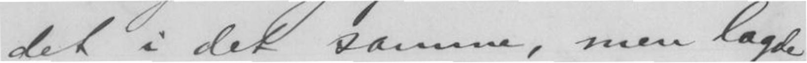det i det samme, men lagde
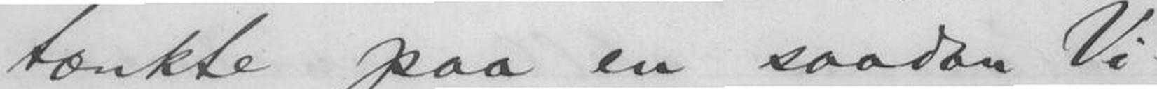tænkte paa en saadan Vi-
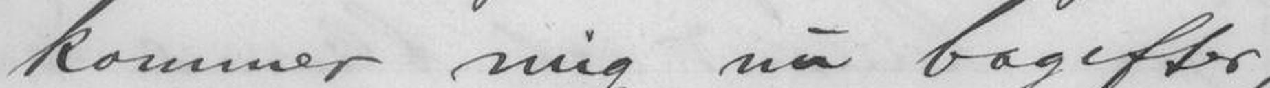kommer mig nu bagefter,
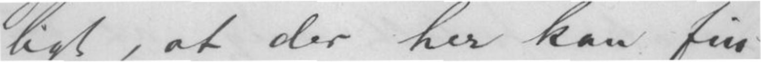ligt, at der her kan fin
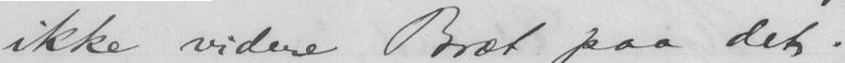ikke videre Bræt paa det.
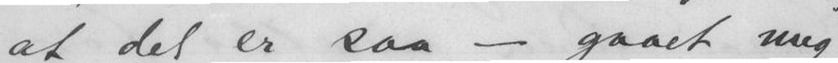at det er saa - gaaet meg
